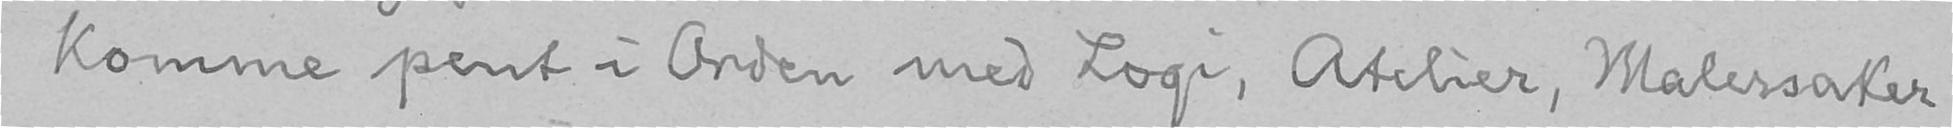komme pent i Orden med Logi, Atelier, Malersaker
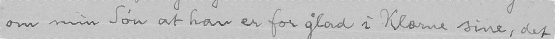om min Søn at han er for glad i Klærne sine, det
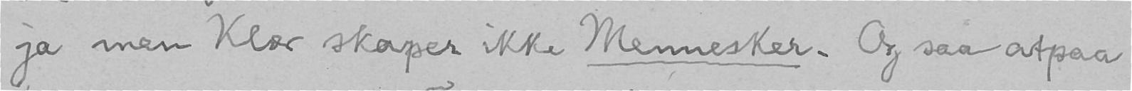ja men Klær skaper ikke Mennesker. Og saa atpaa
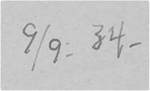9/9. 34.
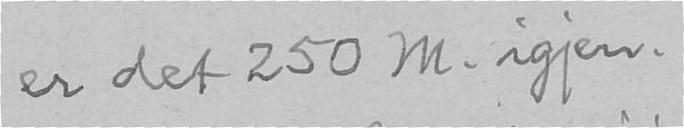er det 250 M. igjen.
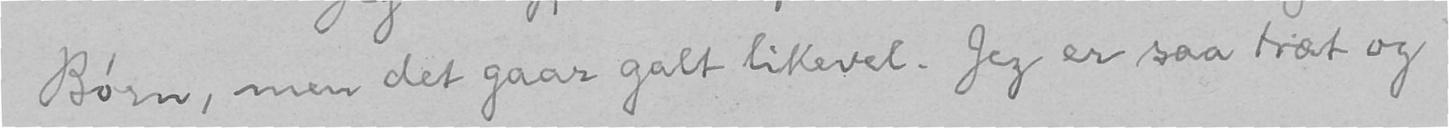Børn, men det gaar galt likevel. Jeg er saa træt og
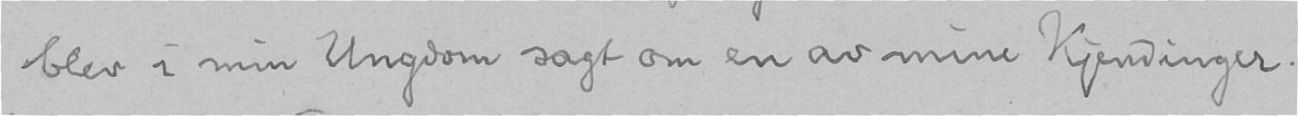blev i min Ungdom sagt om en av mine Kjendinger.
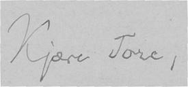Kjære Tore,
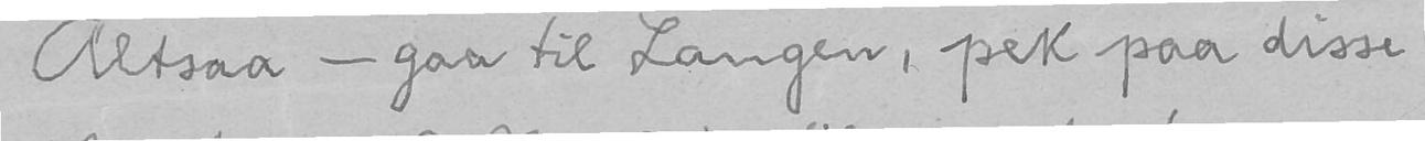Altsaa - gaa til Langen, pek paa disse
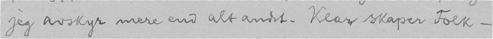jeg avskyr mere end alt andet. Klær skaper Folk -
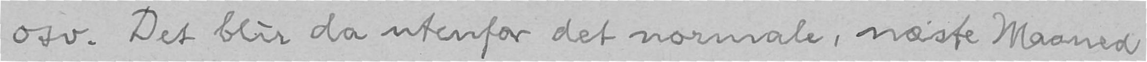osv. Det blir da utenfor det normale, næste Maaned
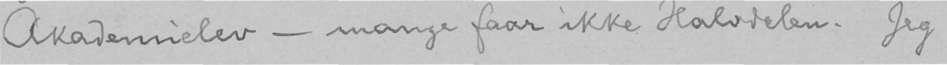Akademielev - mange faar ikke Halvdelen. Jeg
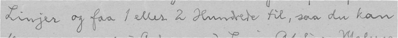Linjer og faa 1 eller 2 Hundrede til, saa du kan
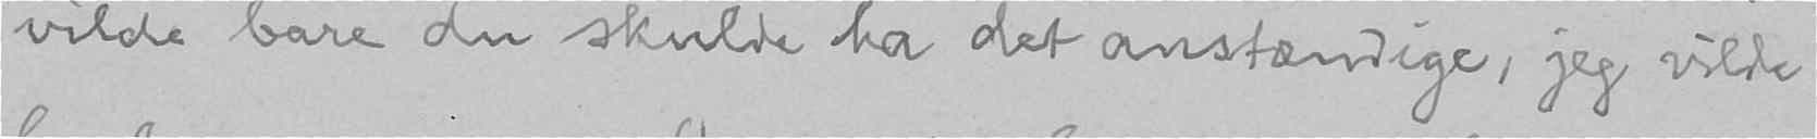vilde bare du skulde ha det anstændige, jeg vilde
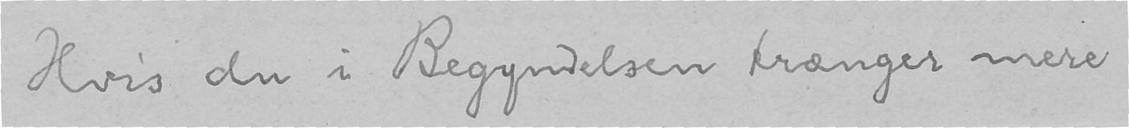Hvis du i Begyndelsen trænger mere
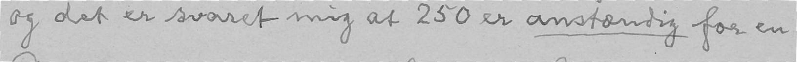og det er svaret mig at 250 er anstændig for en
